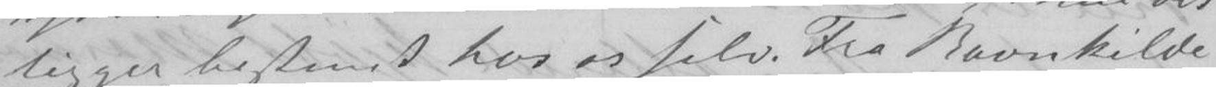ligger bestemt hos os selv. Fra Ravnkilde
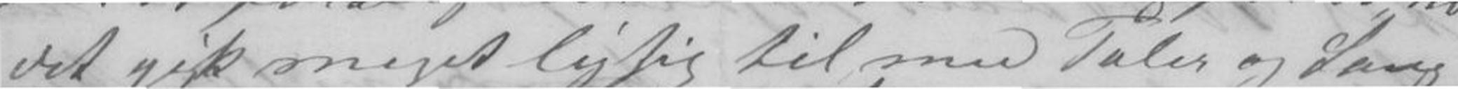det gik meget liflig til med Taler og Sang
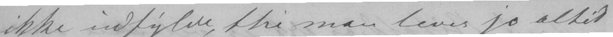ikke indfylde, thi man lever jo altid
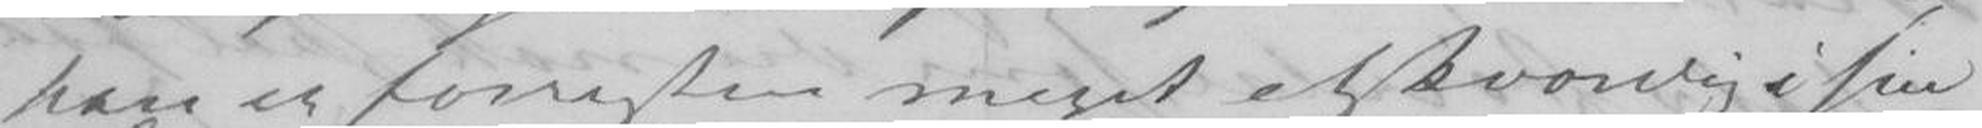han er forresten meget elskværdig i sin
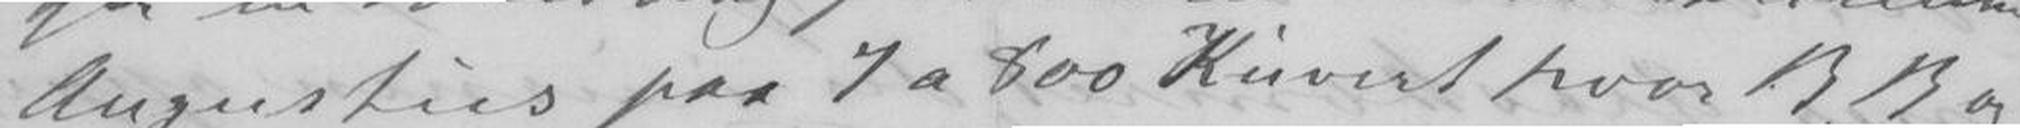Augustus paa 7 a 800 Kuvert hvor B.B og
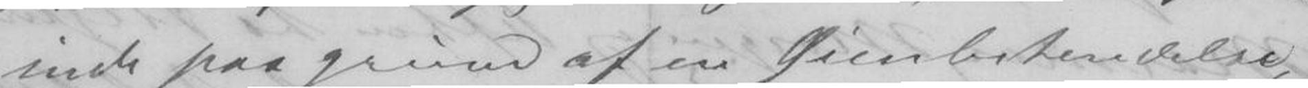inde paa grund af en Øienbetendelse,
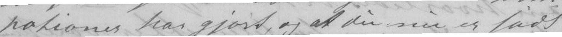potioner har gjort, og at du nu er sadt
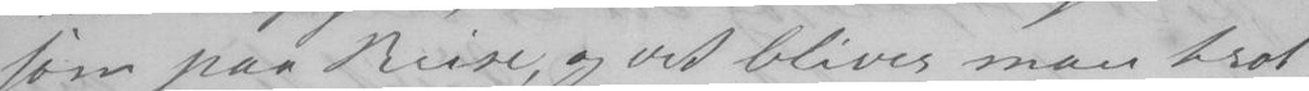som paa Reise, og det bliver man trot
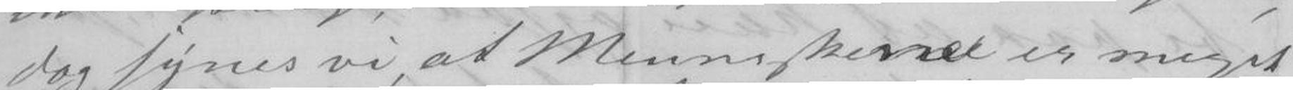dog synes vi, at Menneskerne er meget
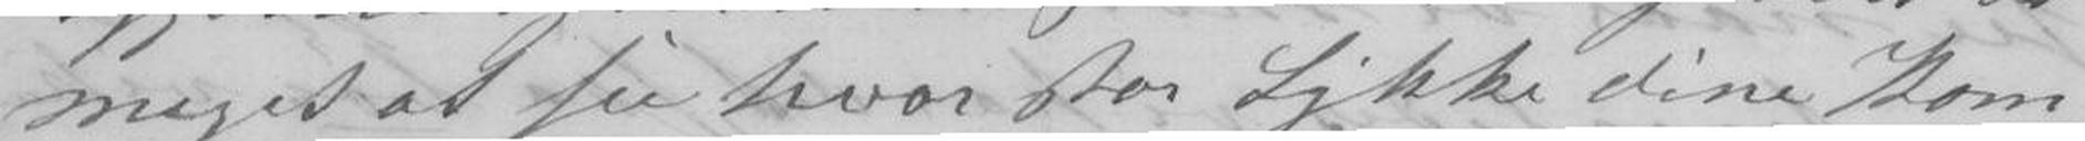meget at see hvor stor Lykke dine Kom-
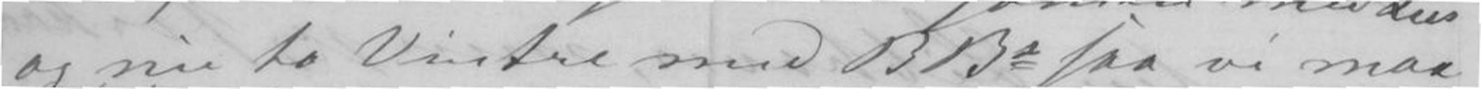og nu to Vintre med B Bs saa vi maa
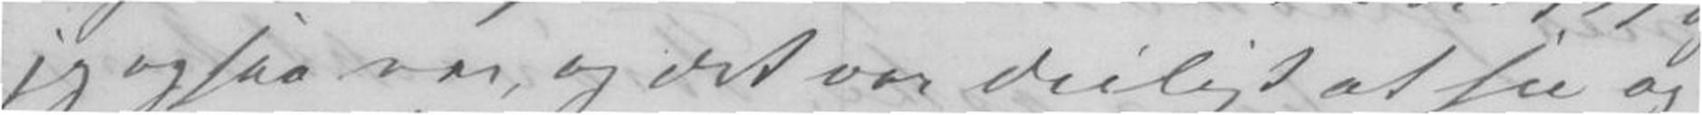jeg ogsaa var, og det var deiligt at see og
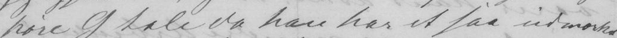høre G. tale da han har et saa udmærket
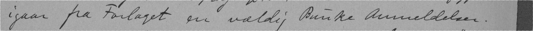igaar fra Forlaget en vældig Bunke Anmeldelser.
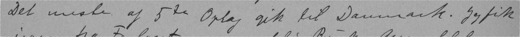Det meste af 5te Oplag gik til Danmark. Jeg fik
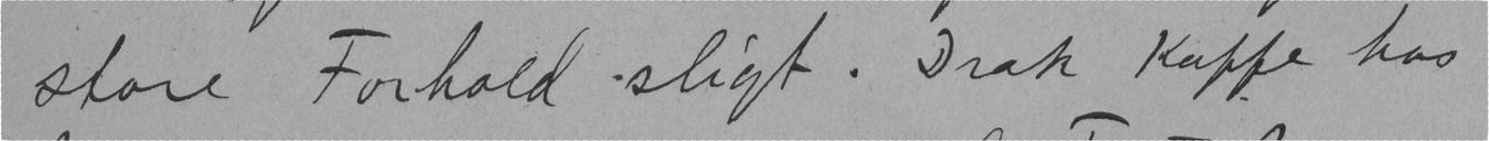store Forhold sligt. Drak Kaffe hos
